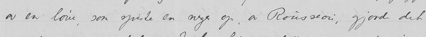av en løve, som spiste en neger op, av Rousseau, gjorde det
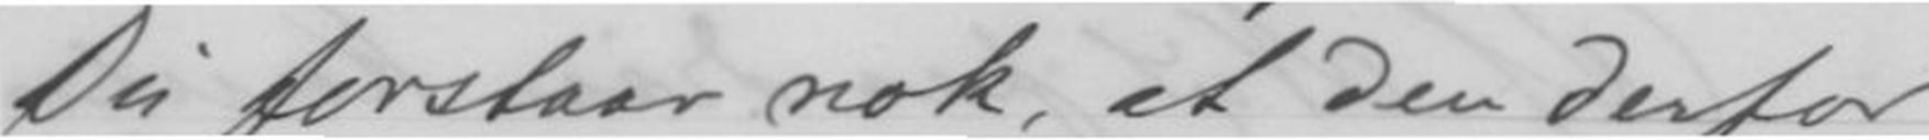Du forstaar nok, at den derfor
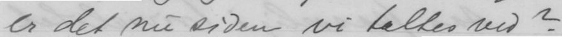er det nu siden vi taltes ved?
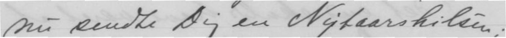nu sendte Dig en Nytaarshilsen;
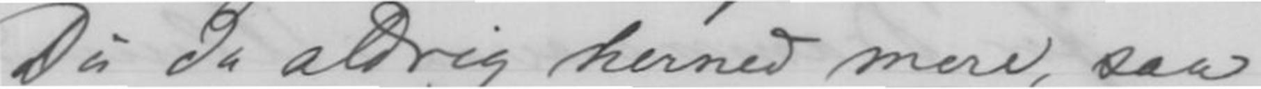Du da aldrig herned mere, saa
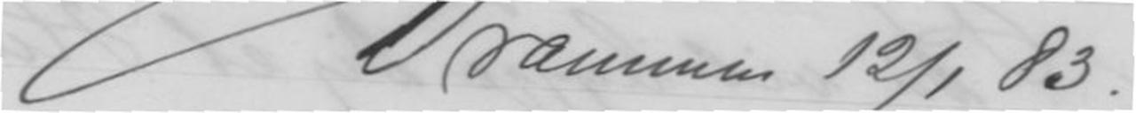Drammen 12/1 83.
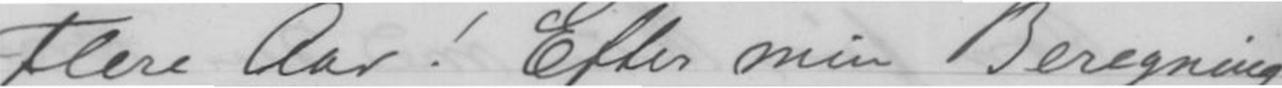Flere Aar! Efter min Beregning
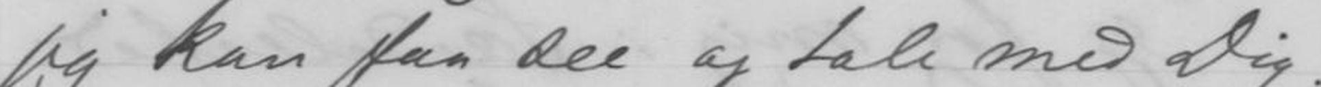jeg kan faa see og tale med Dig.
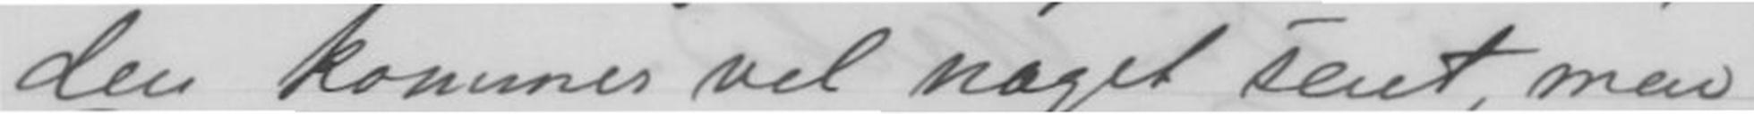den kommer vel noget sent, men
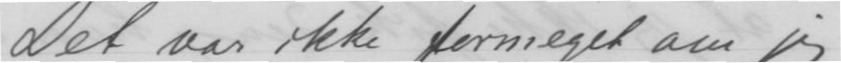Det var ikke formeget om jeg
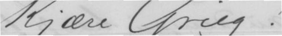Kjære Grieg!
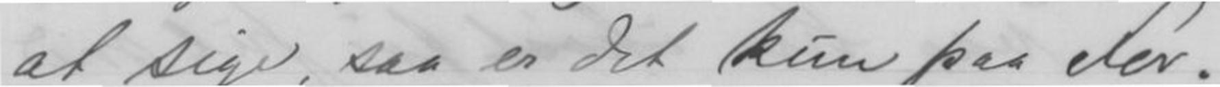at sige saa er det kun paa For-
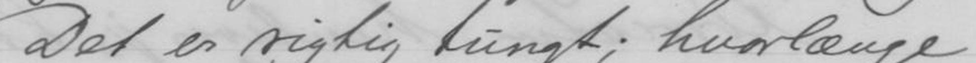Det er rigtig tungt; hvorlænge
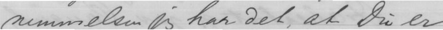nemmelsen jeg har det at Du er
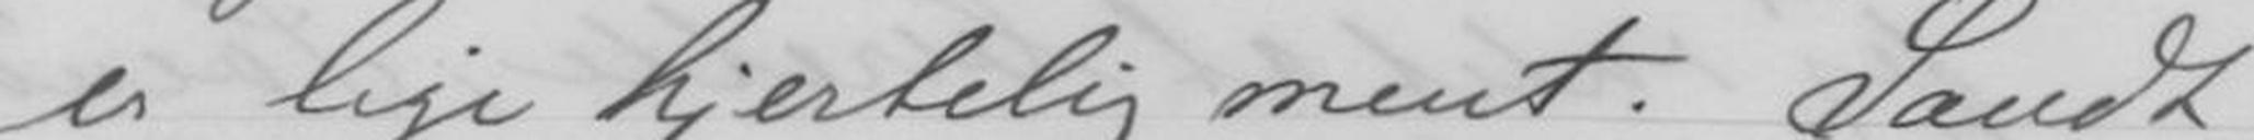er lige hjertelig ment. Sandt
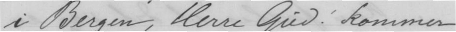i Bergen, Herre Gud kommer
